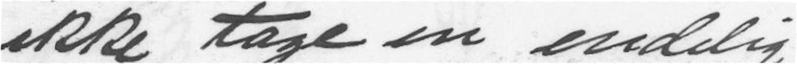ikke tage en endelig
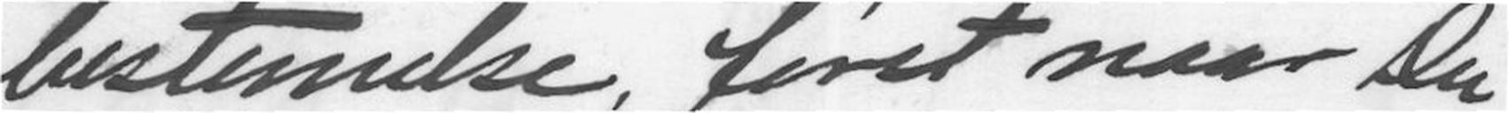bestemelse, først naar Du
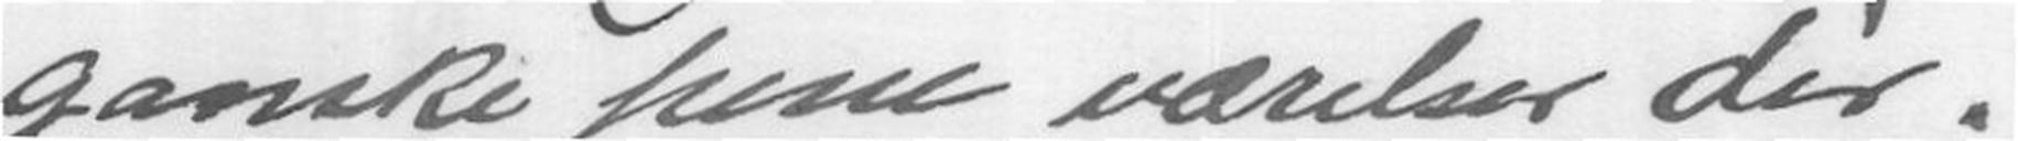ganske pene værelser dèr.
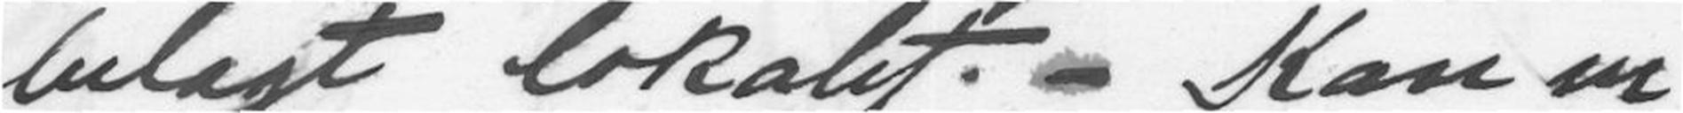belagt lokalet. - Kan vi
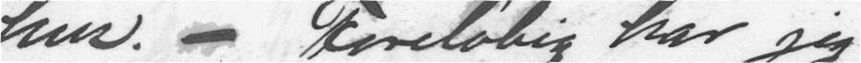hus. - Foreløbig har jeg
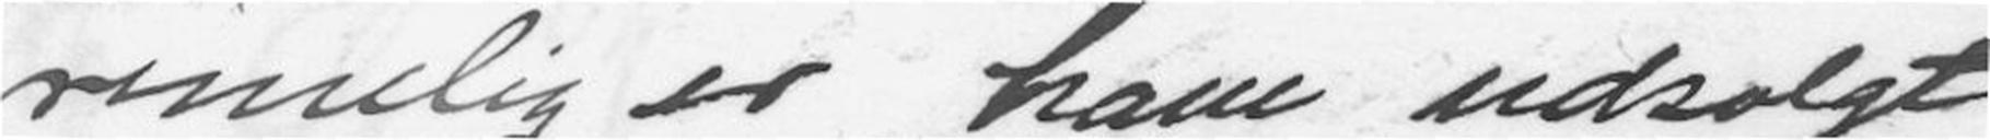rimelig er have udsolgt
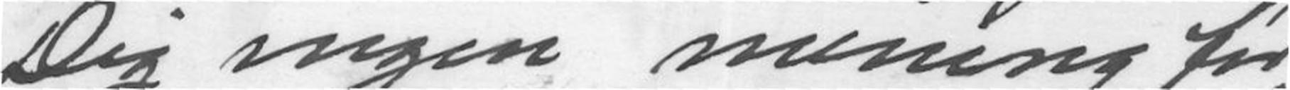Dig ingen mening før.
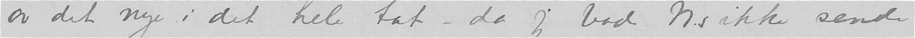av det nye i det hele tat -da jeg bad N.s ikke sende
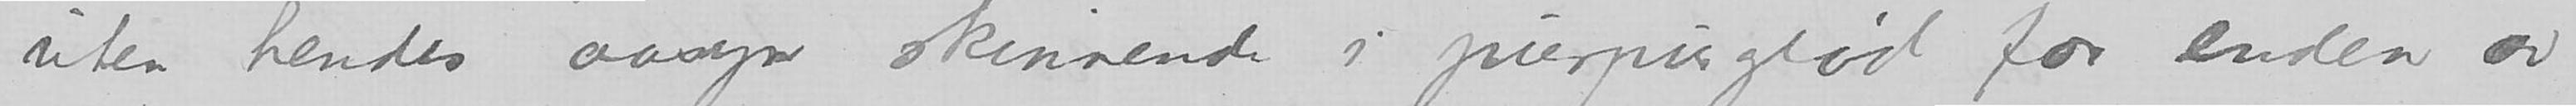uten hendes aasyn skinnende i purpurglød for enden av
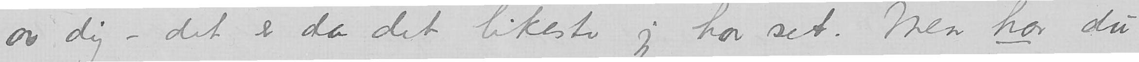av dig -det er da det likeste jeg har set. Men har du
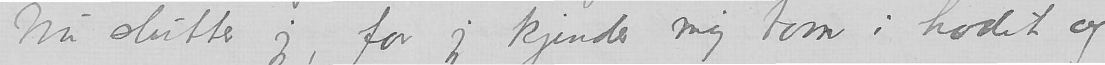Nu slutter jeg, for jeg kjender mig tom i hodet og
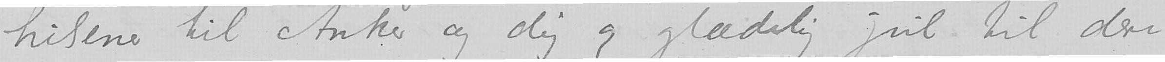hilsener til Anker og dig og glædelig jul til dere
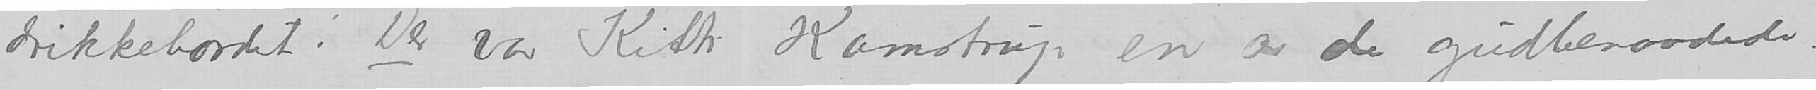drikkebordet! Der var Kitti Kamstrup en av de gudbenaadede!
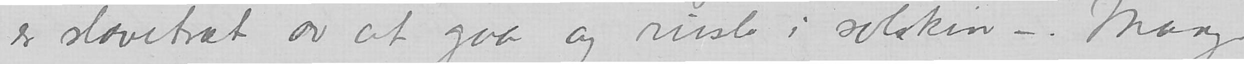er sløvetræt av at gaa og rusle i solskin - Mange
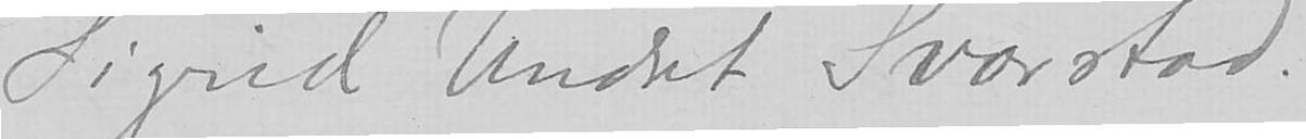Sigrid Undset Svarstad
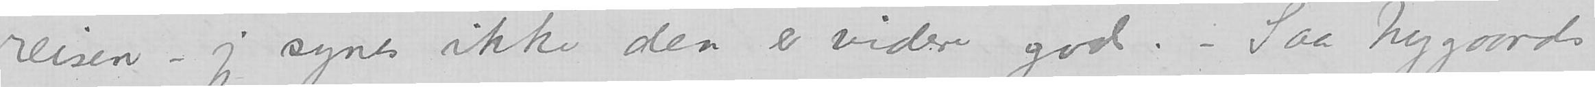reisen – jeg synes ikke den er videre god. – Saa Nygaards
